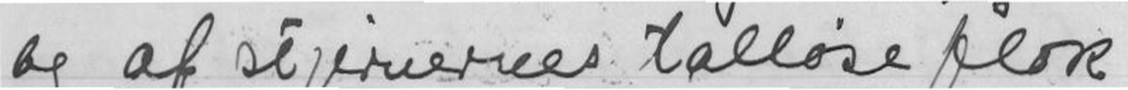og af stjernernes talløse flok
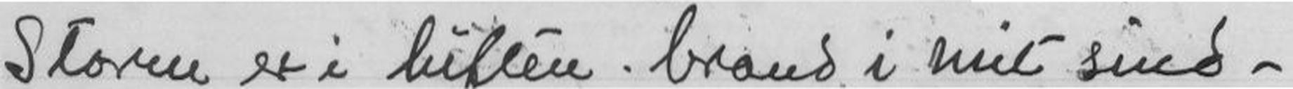Storm er i luften brand i mit sind -
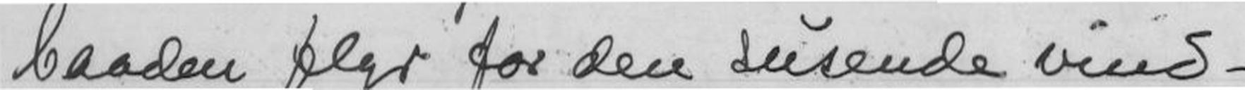baaden flyr for den susende vind -
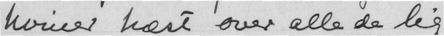hviner hæst over alle de lig
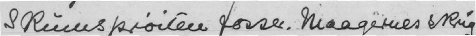Skumsprøiten fosser. Maagernes skrig
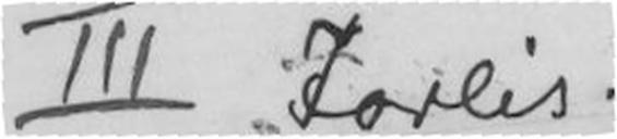III Forlis.
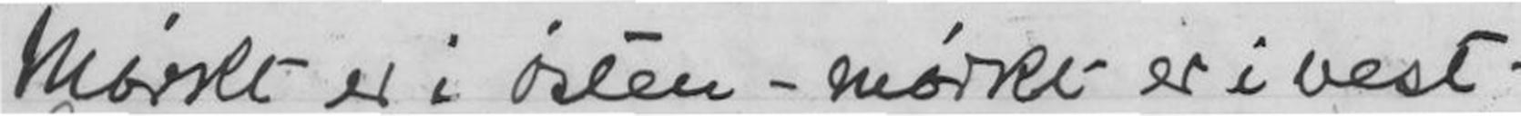Mørkt er i østen - mørkt er i vest -
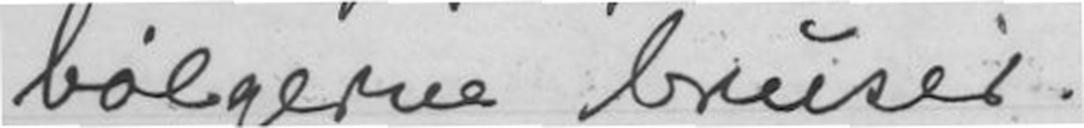bølgerne bruser.
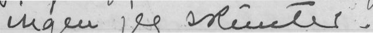ingen jeg skimter.
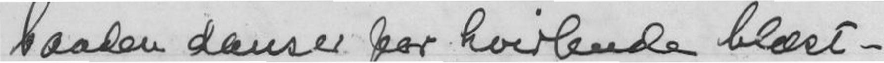baaden danser for hvirlende blæst -
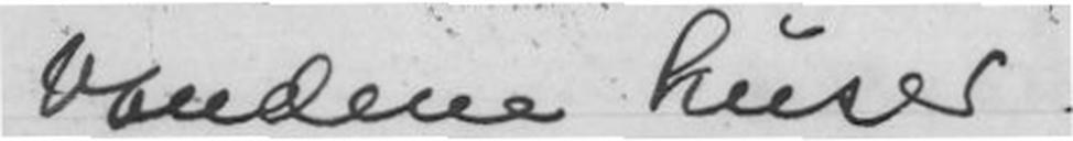vandene huser.
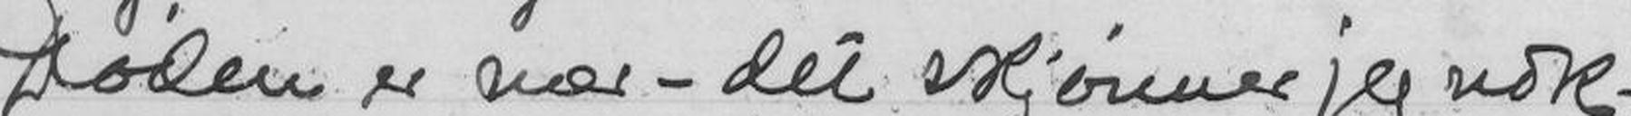Døden er nær - det skjønner jeg nok -
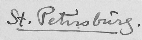St. Petersburg.
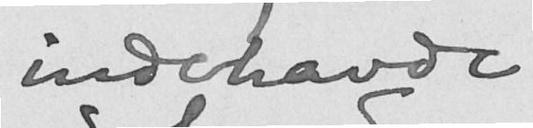indehavde
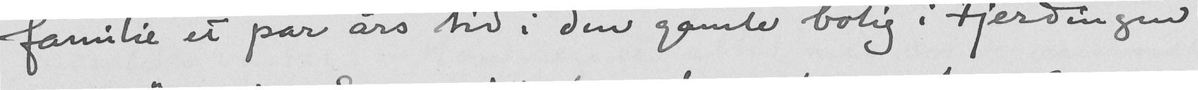familie et par års tid i den gamle bolig i Fjerdingen
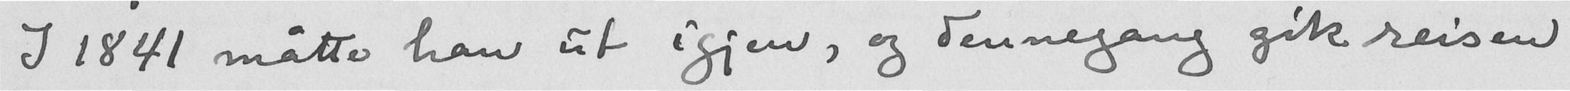I 1841 måtte han ut igjen, og dennegang gik reisen
